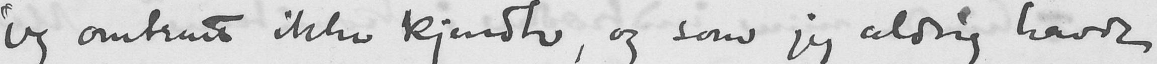jeg omtrent ikke kjendte, og som jeg aldrig havde
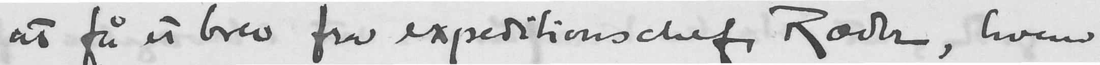at få et brev fra expeditionschef Ræder, hvem
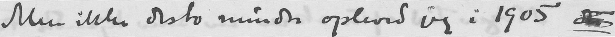Men ikke desto mindre opleved jeg i 1905 det
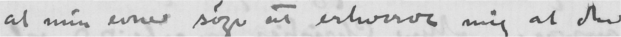al min evne søge at erhverve mig al den
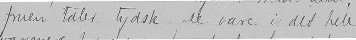fruen taler tydsk. De vare i det hele
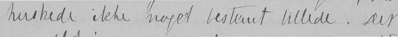huskede ikke noget bestemt billede. Det
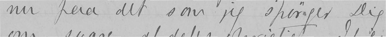nu paa det som jeg spørger Dig
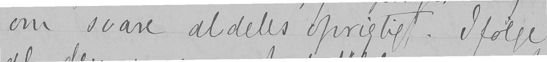om svare aldeles oprigtigt. Ifølge
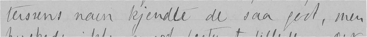terssens navn kjendte de saa godt, men
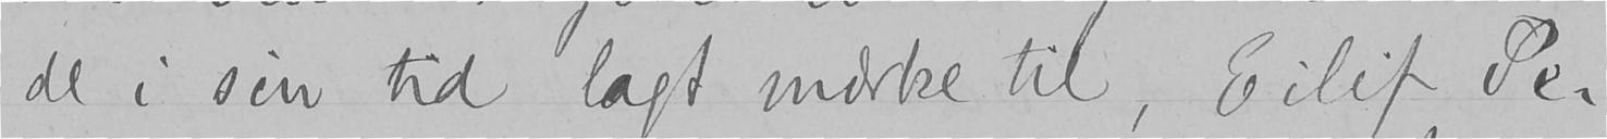de i sin tid lagt mærke til, Eilif Pe-
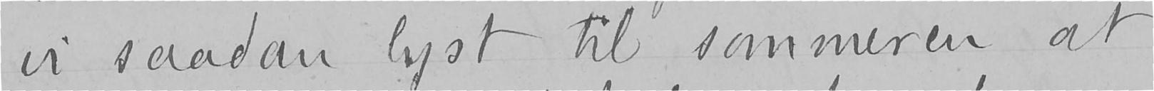vi saadan lyst til sommeren at
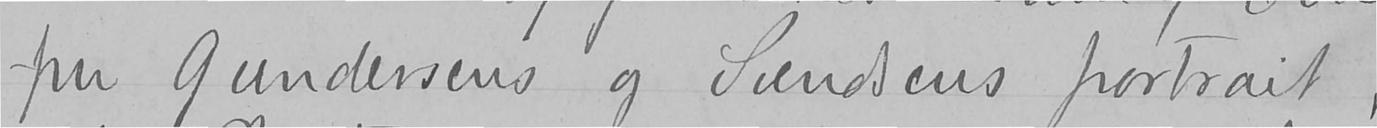fru Gundersens og Sundsens portrait,
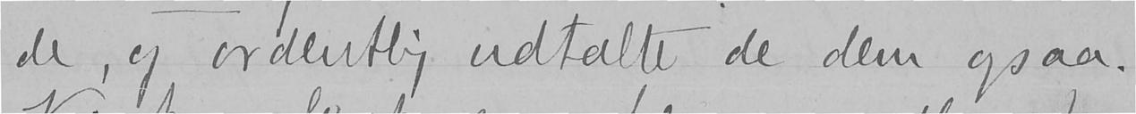de, og ordentlig udtalte de dem ogsaa.
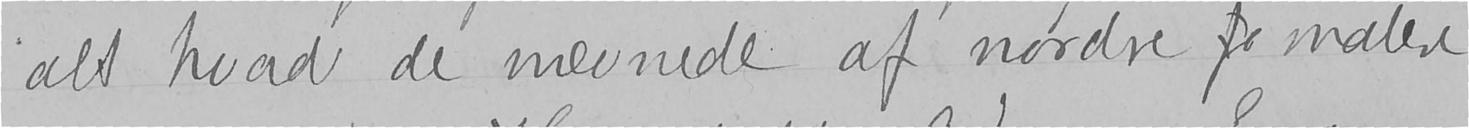alt hvad de nævnede af nordre fo malere
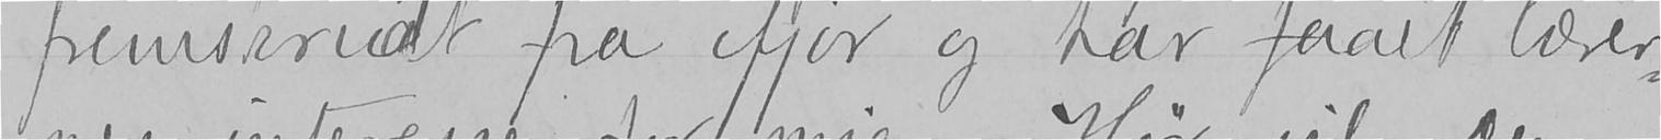fremskridt fra ifjor og har faaet lærer-
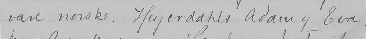vare norske. Heyerdahls Adam og Eva,
